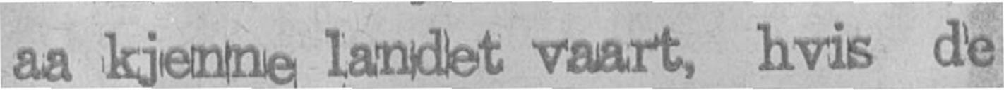aa kjenne landet vaart, hvis de
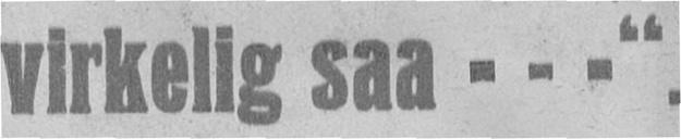virkelig saa...".
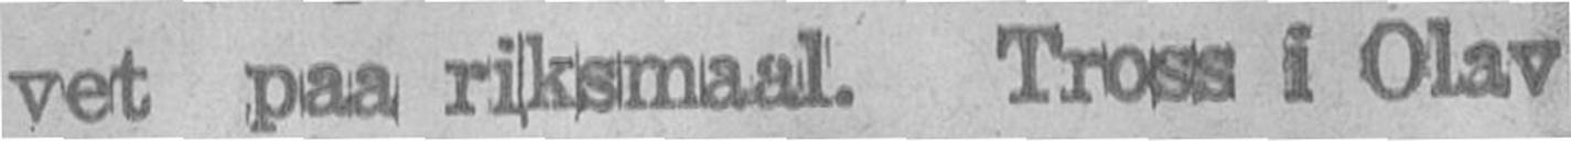vet paa riksmaal. Tross i Olav
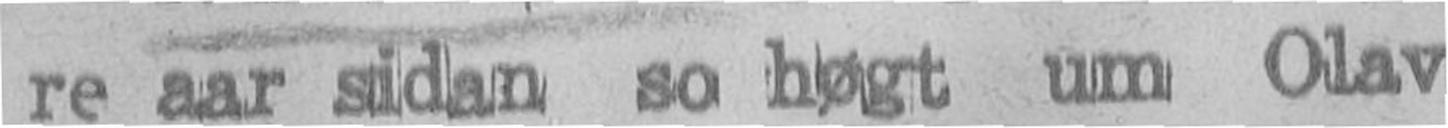re aar sidan so hogt um Olav
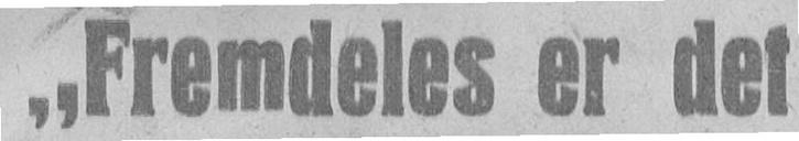„Fremdeles er det
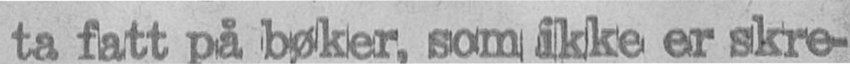ta fatt på bøker, som ikke er skre-
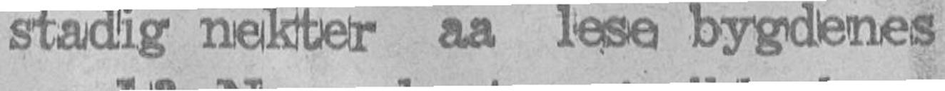stadig nekter aa lese bygdenes
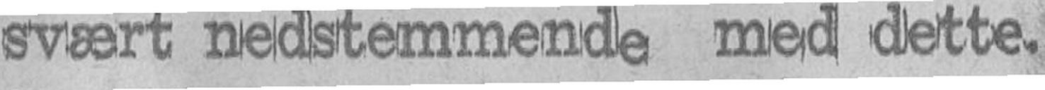svært nedstemmende med dette.
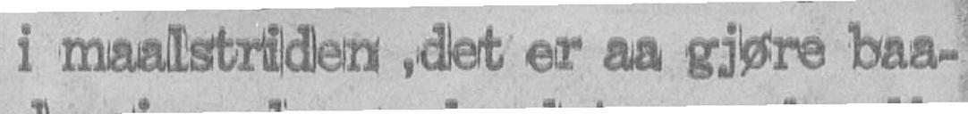i maalstriden ,det er aa gjøre baa-
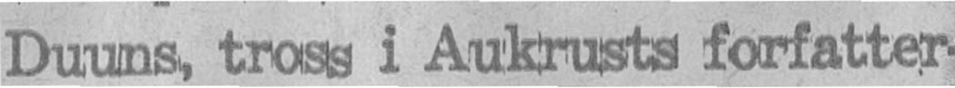Duuns, tross i Aukrusts forfatter
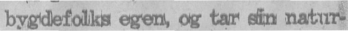bygdefolks egen, og tar sin natur-
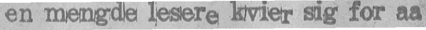en mengde lesere kvier sig for aa
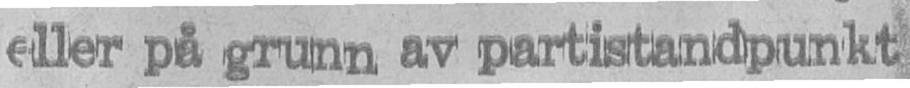eller på grunn av partistandpunkt
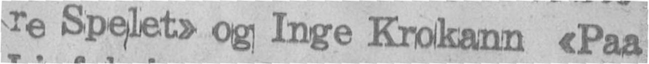re Spelet» og Inge Krokann «Paa
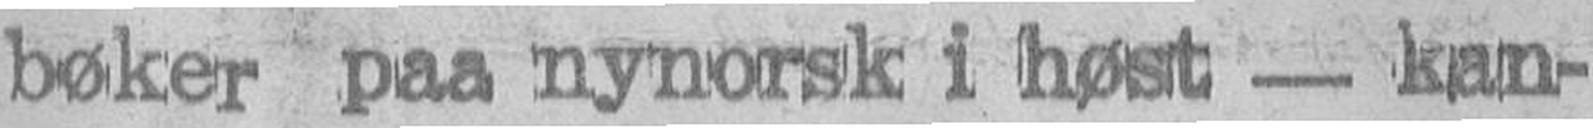boker paa nynorsk i høst — kan-
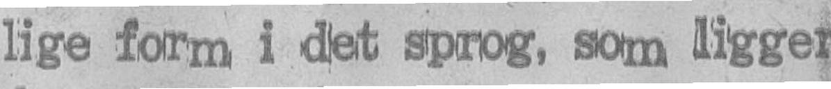lige form i det sprog, som ligger
